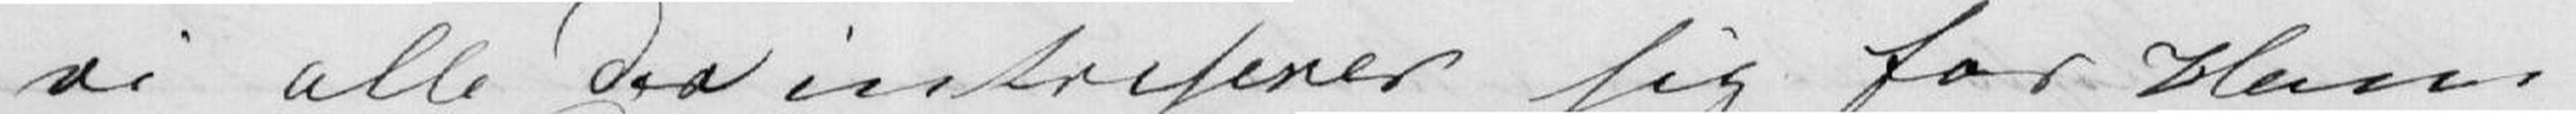vi alle da intreserer sig for Ham.
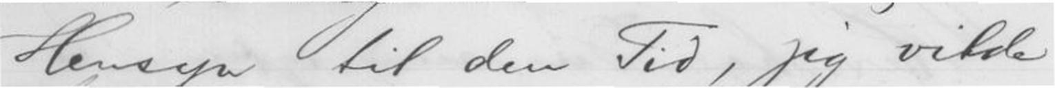Hensyn til den Tid, jeg vilde
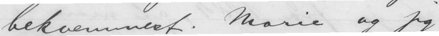bekvemmest. Marie og jeg
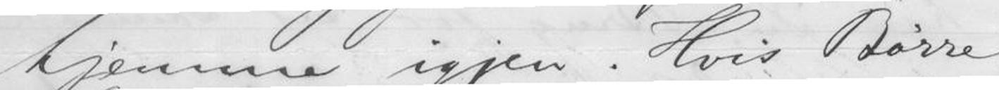hjemme igjen. Hvis Børre
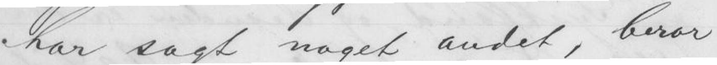har sagt noget andet, beror
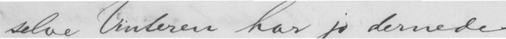selve Vinteren har jo dernede
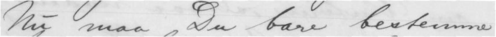Nu maa Du bare bestemme
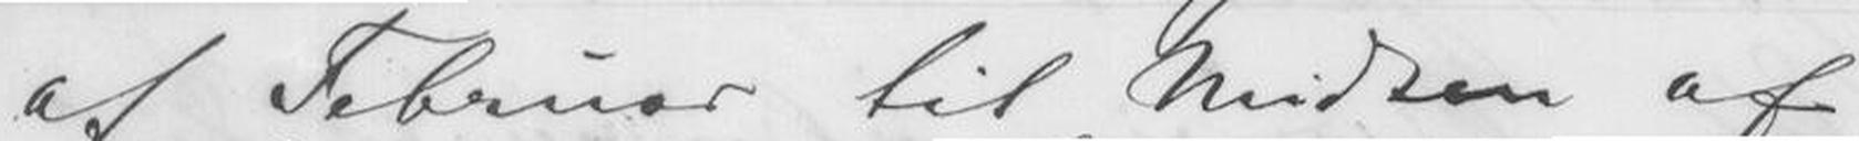af Februar til Midten af
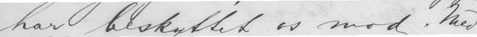har beskyttet os mod. Med
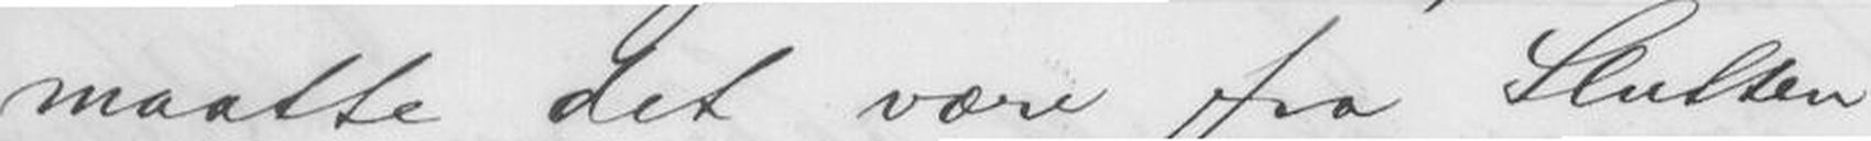maatte det være fra Slutten
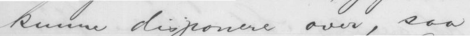kunne disponere over, saa
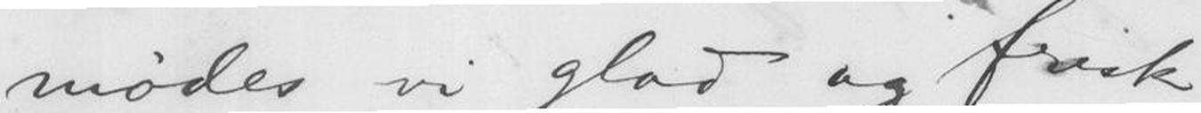mødes vi glad og frisk
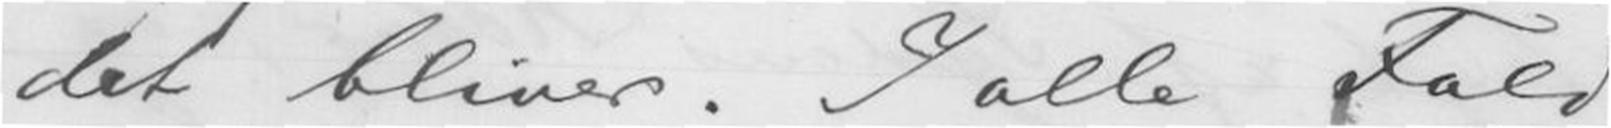det bliver. I alle Fald
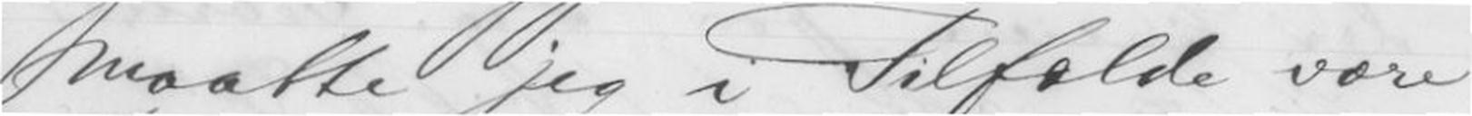maatte jeg i Tilfælde være
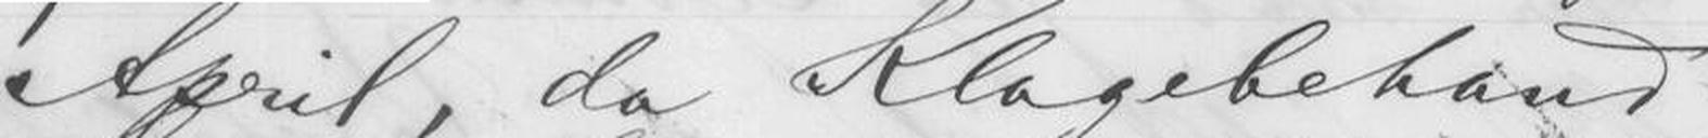April, da Klagebehand-
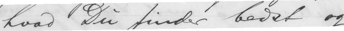hvad Du finder bedst og
